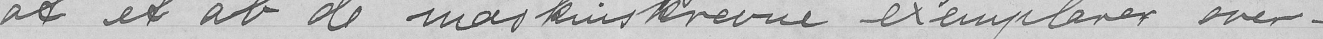at et av de maskinskrevne exemplarer over-
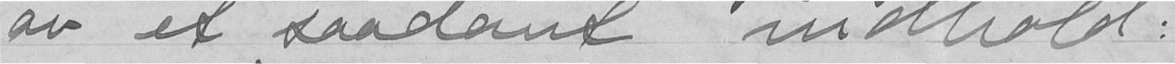av et saadant indhold:
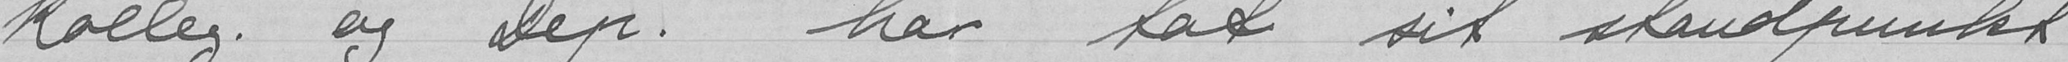Kolleg. og Dep. har tat sit standpunkt
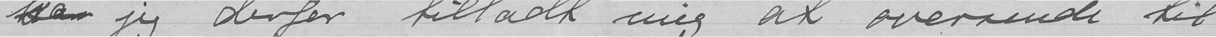har jeg derfor tilladt mig at oversende til
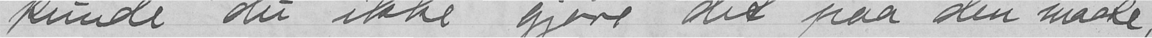Kunde du ikke gjøre det paa den maate,
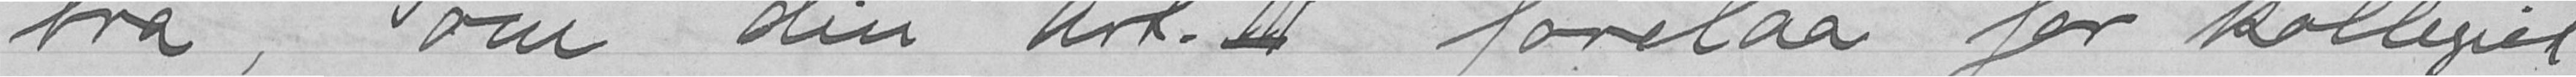bra, om din art. III forelaa for kollegiet
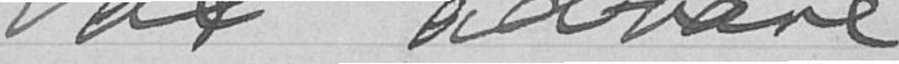at advare
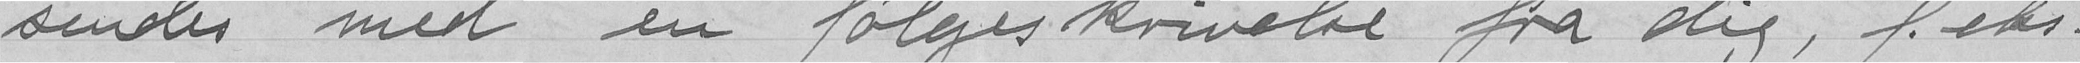sendes med en følgeskrivelse fra dig, f.eks.
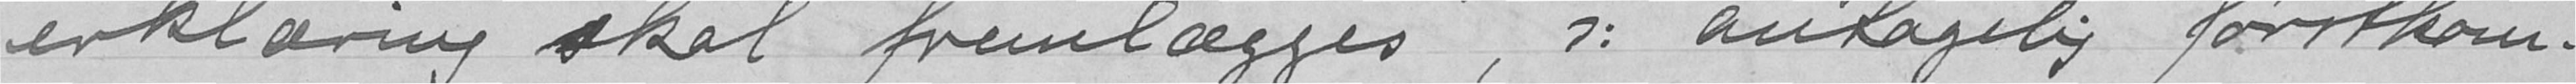erklæring skal fremlægges, : antagelig førstkom-
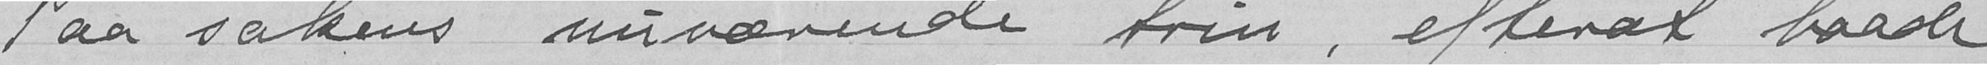Paa sakens nuværende trin, efterat baade
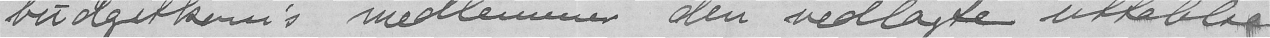budgetkomm's medlemmer den vedlagte uttalelse
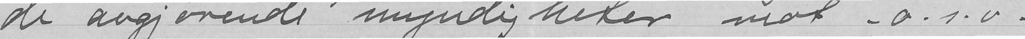de avgjørende myndigheter mot .o.s.v.
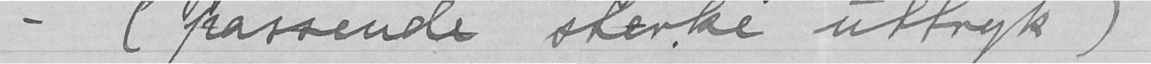- (passende stærke uttryk)
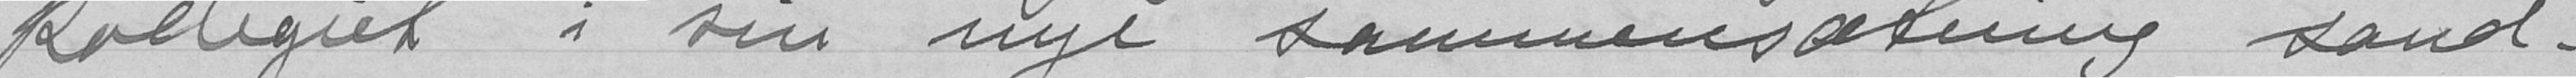kollegiet i sin nye sammensætning sand-
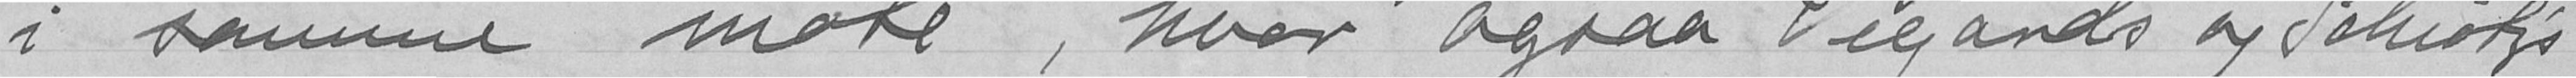i samme møte, hvor ogsaa Vigands og Schiøtz's
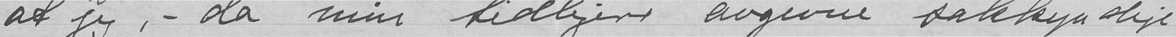at jeg, - da min tidligere angivne sakkyndige
